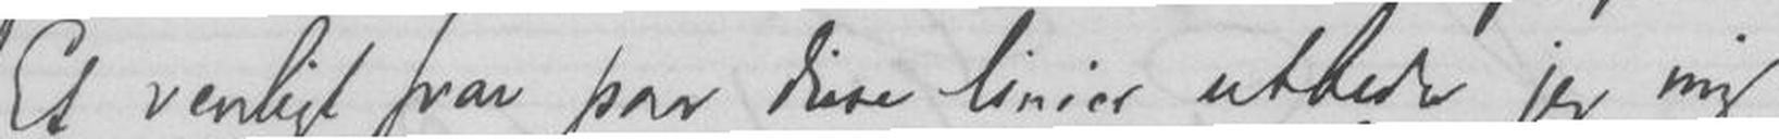Et venligt svar paa disse linier utbeder jeg mig
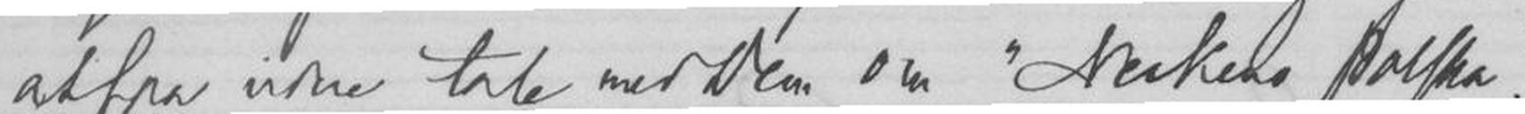at fra videre tale med dem om Neckens polska.
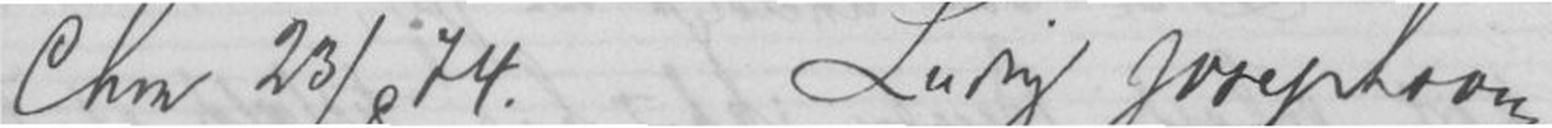Chra. 23/8 74 Ludvig Josephson
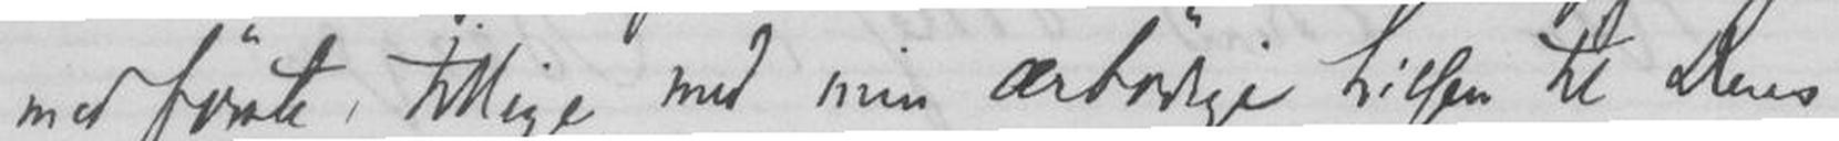med förste, tillige med min Ærbödigste hilsen til Deres
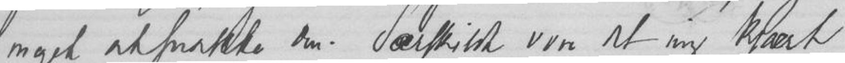meget at snakke om. Særskildt vare det mig kjært
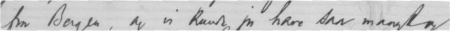fra Bergen, og vi kunde ju have saa mangt og
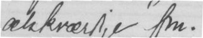ælskværdige fru.
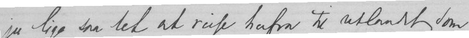ju lige saa let at reise herfra til utlandet som
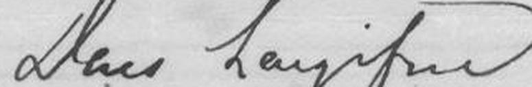Deres hengifne
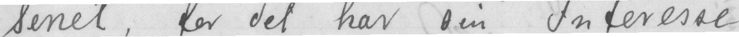senet, for det har sin Interesse
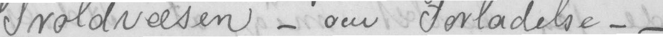Troldvæsen - om Forladelse -
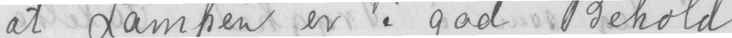at Lampen er i god Behold
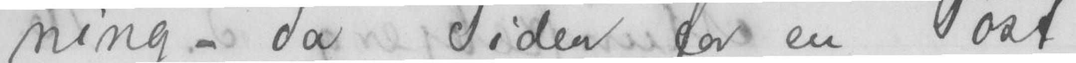ning- da Tiden for en Post
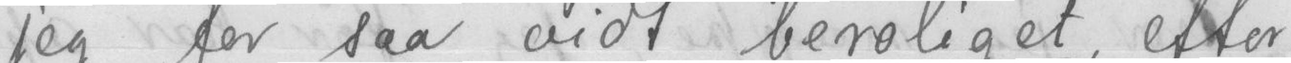jeg for saa vidt beroliget, efter
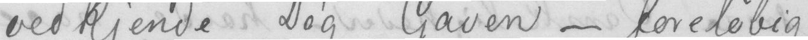vedkjende Dig Gaven - foreløbig -
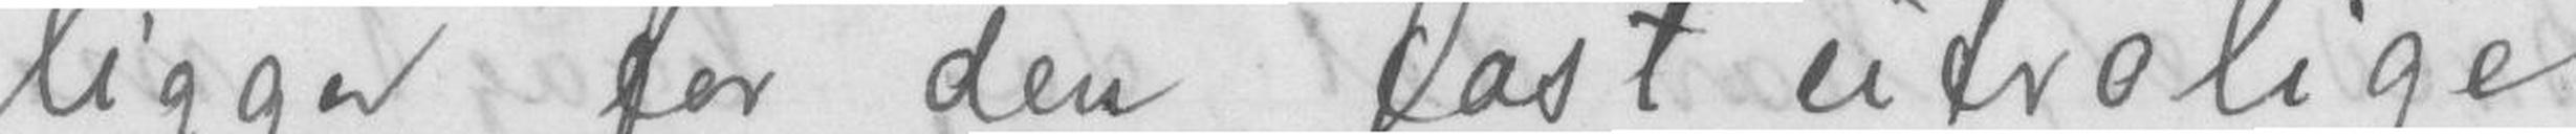ligger for den fast utrolige
